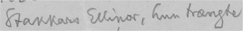Stakkars Ellinor, hun trængte
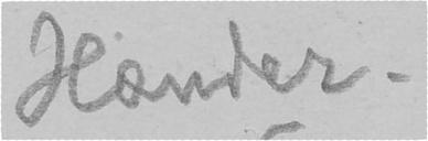Hænder.
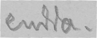endda.
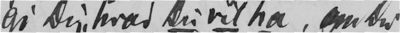gi Dig, hvad Du vil ha, om Du
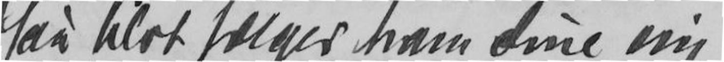saa blot sælger ham dine nye
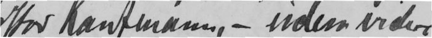forstod Kaufmann, - uden videre
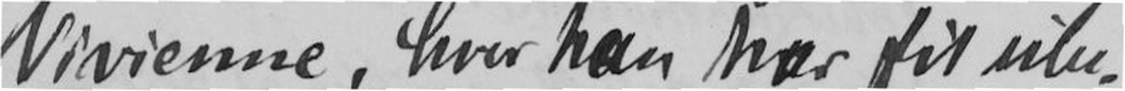Vivienne, hvor han har sit ube-
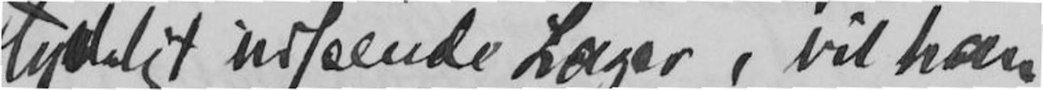tydeligt udseende Lager, vil han
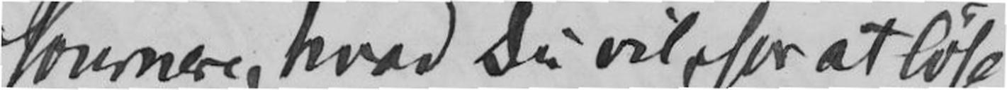soumere, hvad Du vil, for at løse
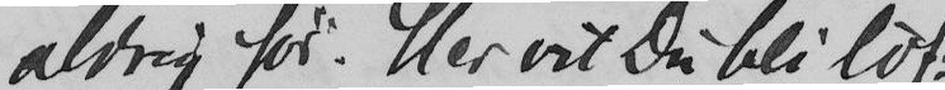aldrig før. Her vil Du bli løf-
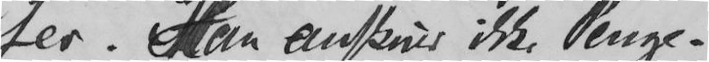ter. Han anskriver ikke Penge-
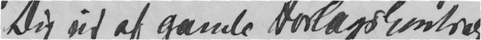Dig ud af gamle Forlagskontrak-
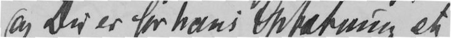og Du er for hans Opfatning et
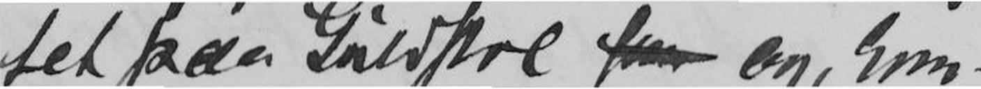tet paa Guldstol og, kom-
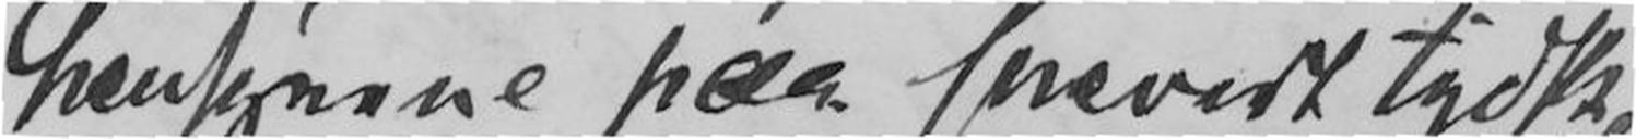hensynene paa snævert tydsk,
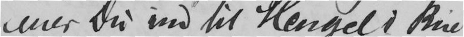mer Du ind til Hengel i Rue
